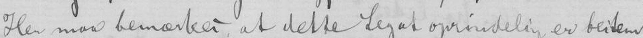Her maa bemærkes at dette Legat oprindelig er bestemt
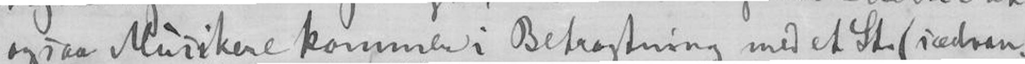ogsaa Musikere kommer i Betragtning med et St. (sædvan
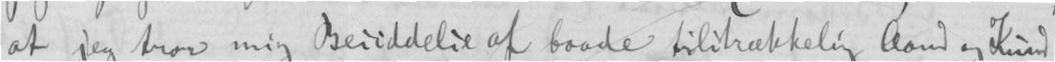at jeg tror mig Besiddelse af baade tilstrækkelig Aand og Kund-
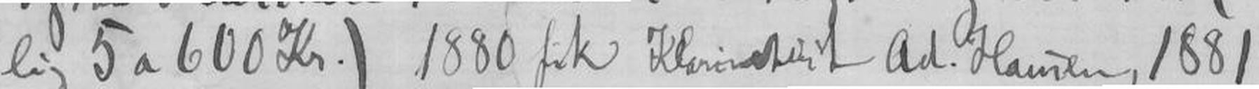lig 5 a 600 Kr.) 1880 fik Klarinitist Ad. Hansen, 1881
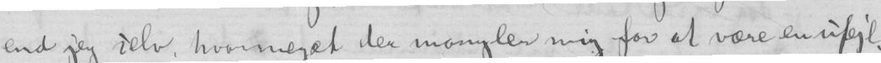end jeg selv hvormeget der mangler mig for at være en ufejl-
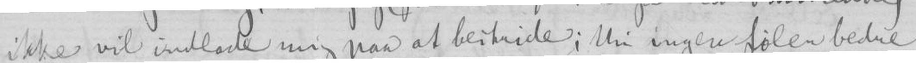ikke vil indlade mig paa at bestride; thi ingen føler bedre
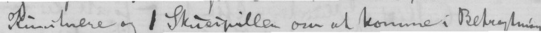Kunstnere og 1 Skuespiller om at komme i Betragtning.
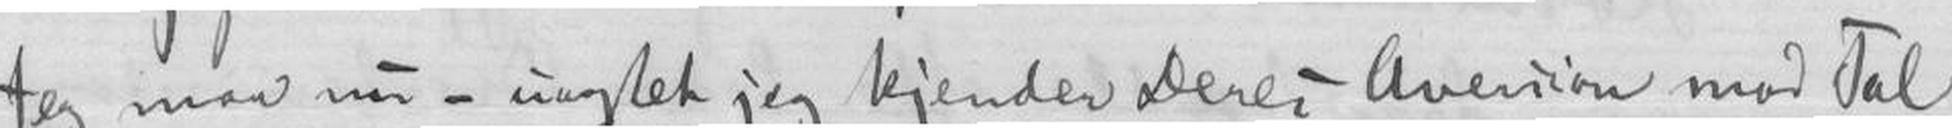Jeg maa nu - uagtet jeg kjender Deres Aversion mod Tal
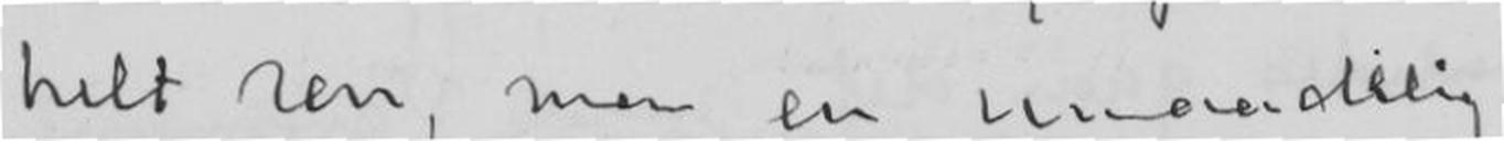helt ren, mer en umaadelig
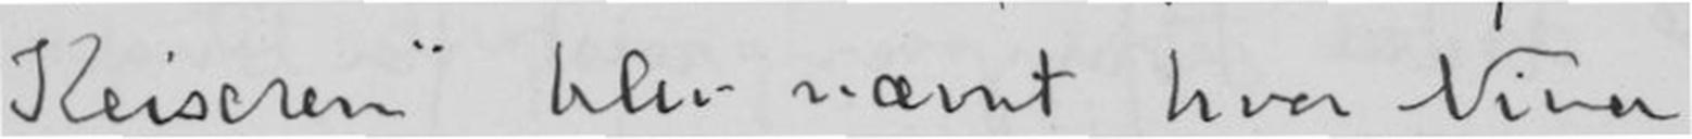"Keiseren" blev nævnt hvor Nina
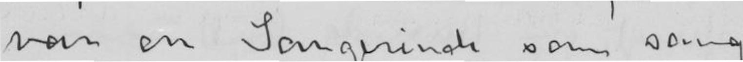var en Sangerinde som sang.
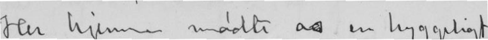Her hjemme mødte os en hyggeligt
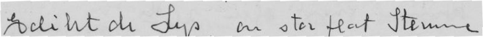Ediht de Lys, en stor flot Stemme
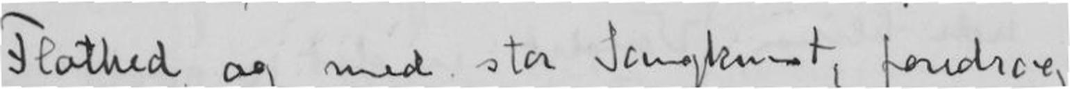Flothed, og med stor Sangkunst, foredrog
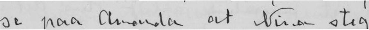se paa Amanda at Nina steg
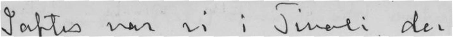Iaftens var vi i Tivoli, der
